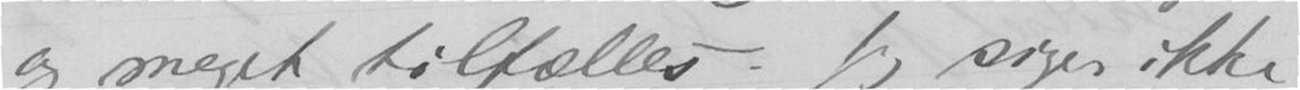og meget tilfælles. Jeg siger ikke
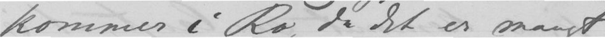kommer i Ro, da det er mangt
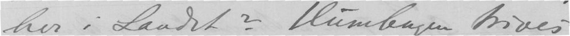her i Landet? Humbugen trivés
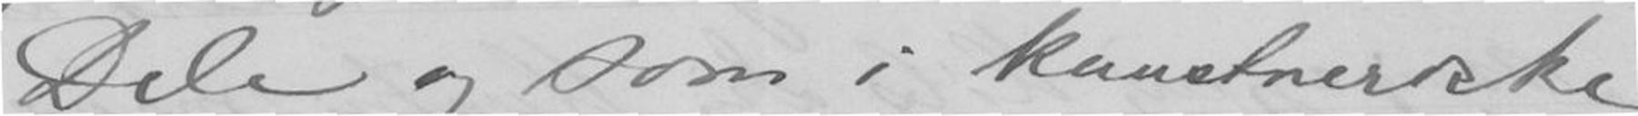Dele og som i kunstnerske
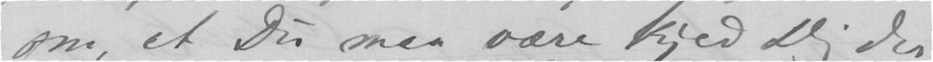om at Du maa være kjed Dig
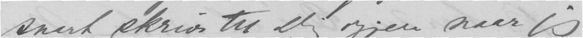snart skrive til Dig igjen naar jeg
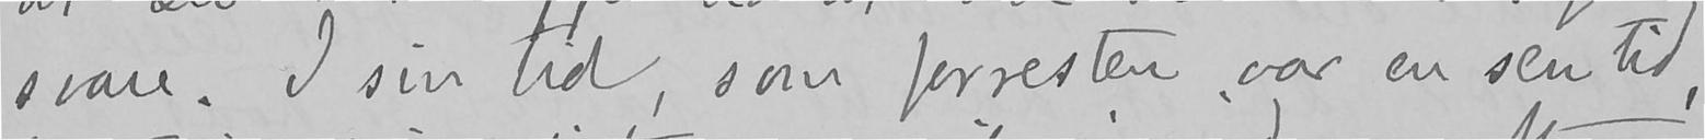svare. I sin tid, som forresten var en sen tid,
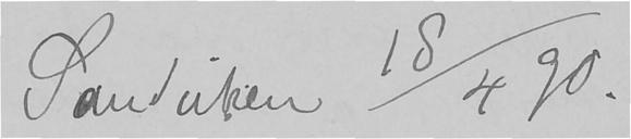Sandviken 18/4 90.
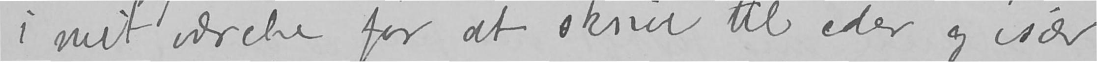i mit værelse for at skrive til eder og især
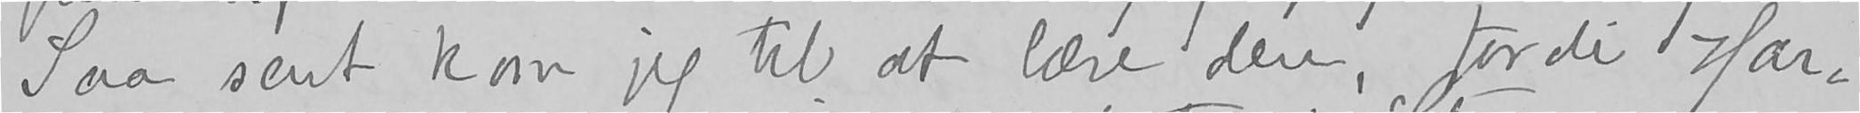Saa sent kom jeg til at læse den, fordi Har-
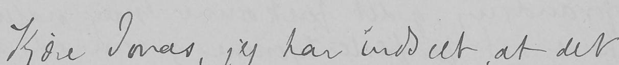Kjære Jonas, jeg har indseet, at det
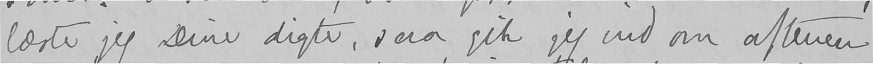læste jeg dine digte, saa gik jeg ind om aftenen
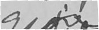gjøre
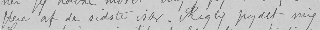flere af de sidste især. Rigtig frydet mig.
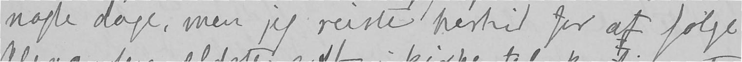nogle dage, men jeg reiste hertil for af følge
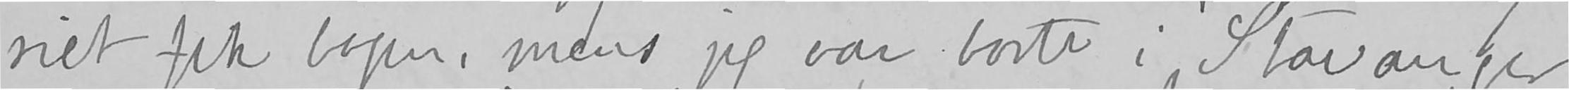riet fik bogen, mens jeg var borte i Stavanger
